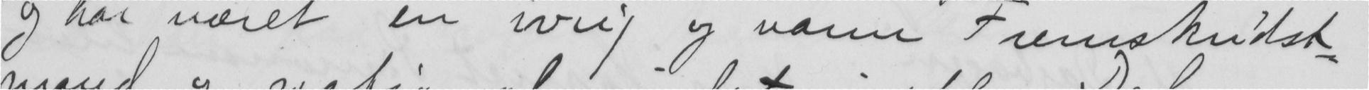og har været en ivrig og varm Fremskridst-
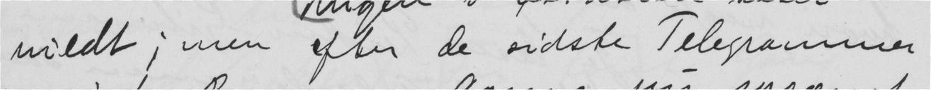vildt; men efter de sidste Telegrammer
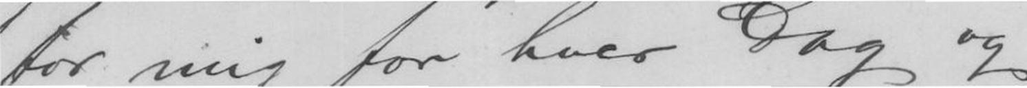for mig for hver Dag og
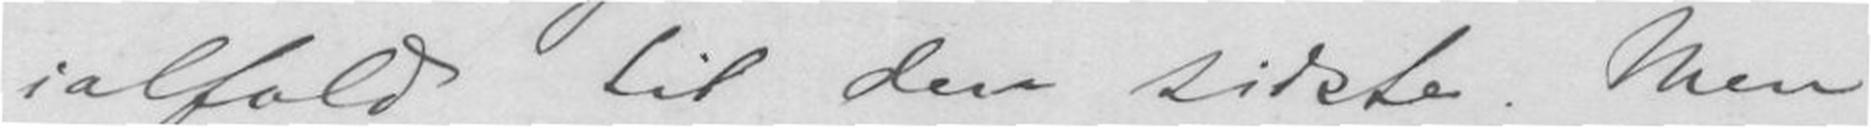ialfald til den sidste. Men
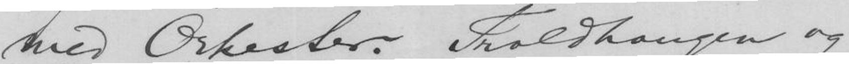med Orkester. Troldhaugen og
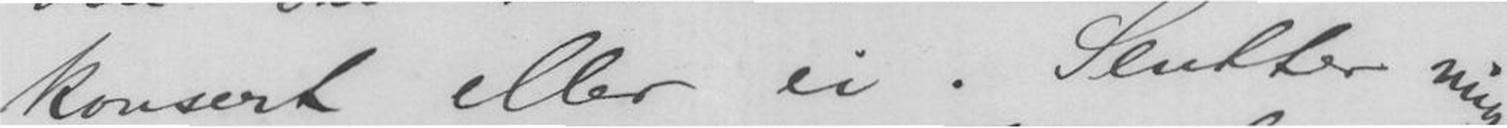konsert eller ei. Slutter mig
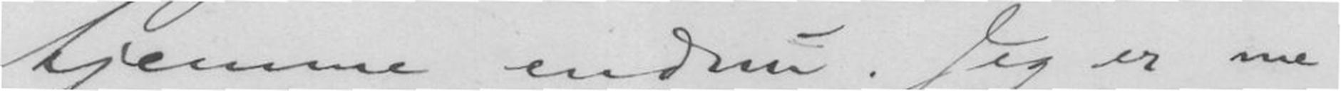hjemme endnu. Jeg er me-
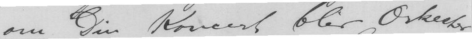om Din Koncert blir Orkester
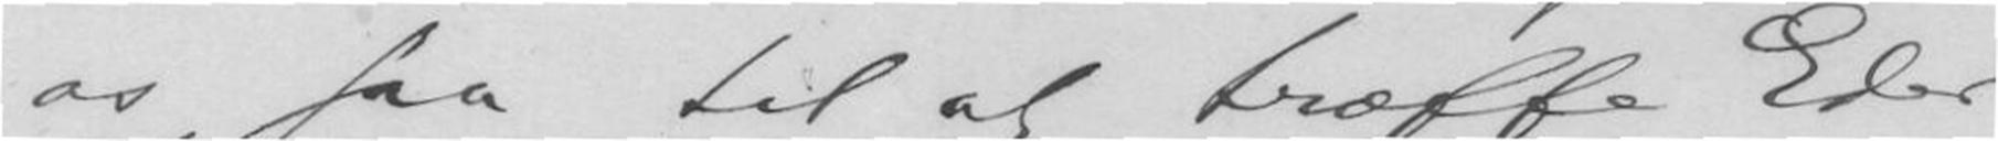os saa til at træffe Eder
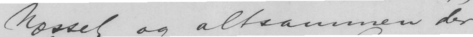Næsset og Altsammen der
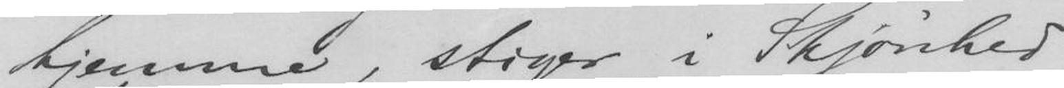hjemme, stiger i Skjønhed
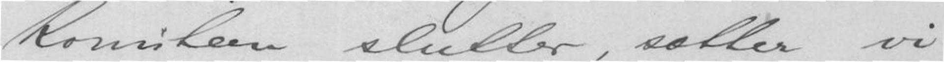Komiteen slutter, sætter vi
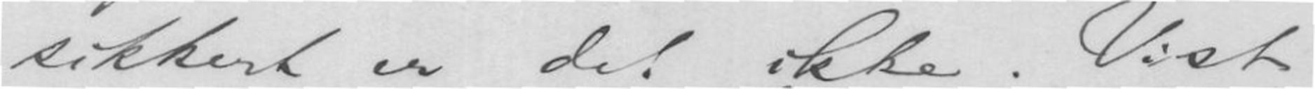sikkert er det ikke. Vist
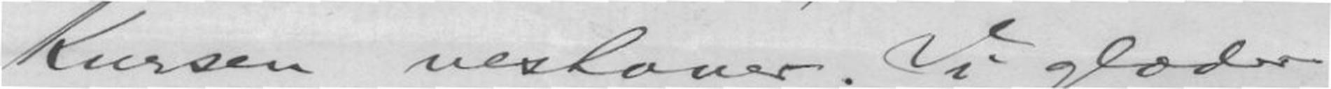Kursen vestover. Vi glæder
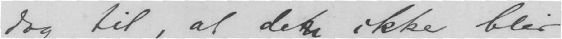dog til, at den ikke blir
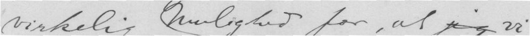virkelig Mulighed, at jeg vi
